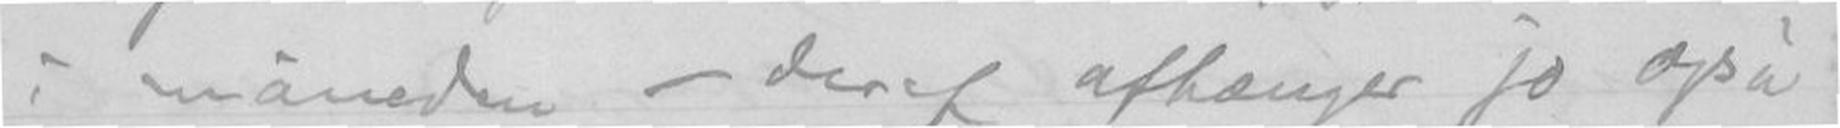i måneden - deraf afhænger jo også
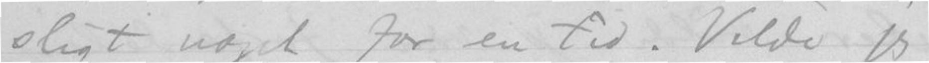sligt noget for en tid. Vilde jeg
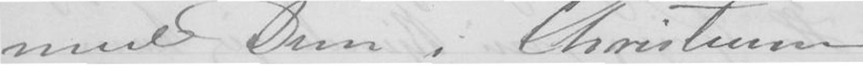med Dem i Christiania.
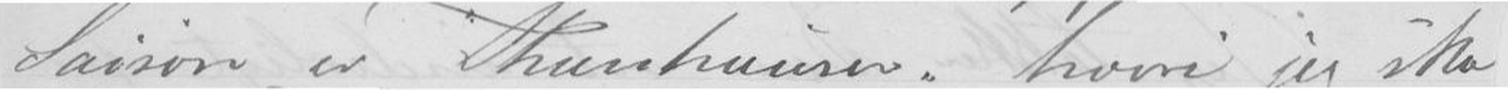Saison er Thanhauser" hvori jeg ska
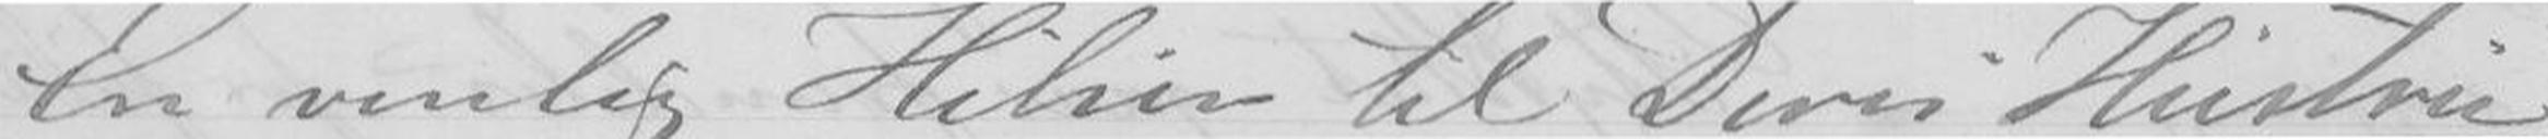En venlig Hilsen til Deres Hustru
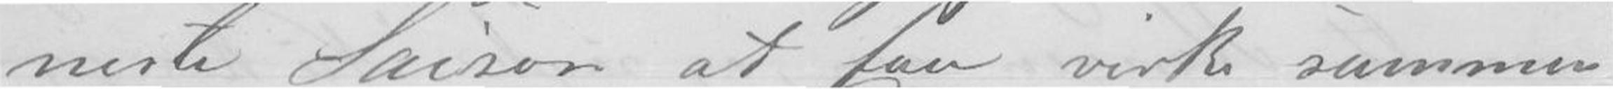neste Saison at faa virke sammen
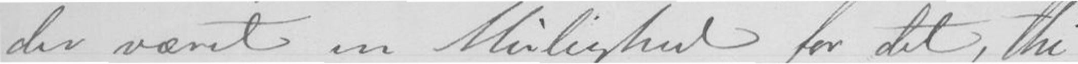der været en Mulighed for det, thi
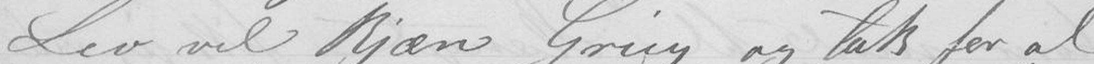Lev vel kjære Grieg og tak for al
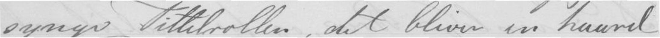synge Tittelrollen, det bliver en haard
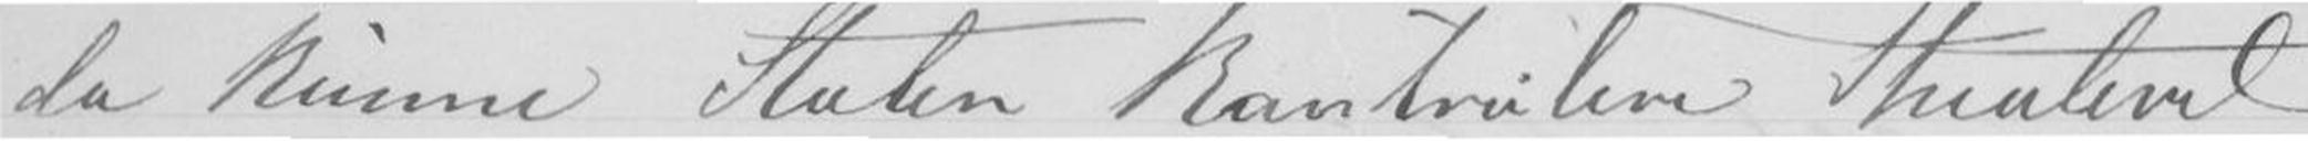da kunne Staten Kontrolere Theateret.
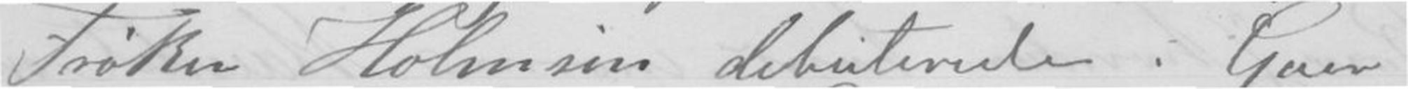Frøken Holmsen debuterte i Gaar
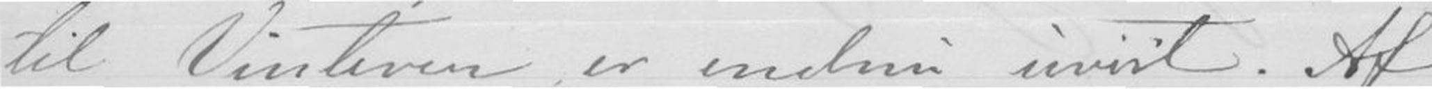til Vinteren er endnu uvist. Af
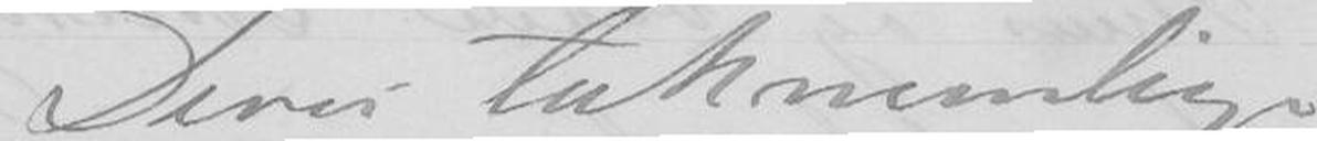Deres taknemlige
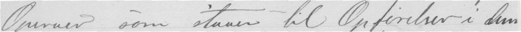Operaer som staaer til Opførelse i dene
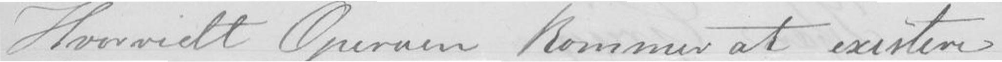Hvorvidt Operaen kommer at existere
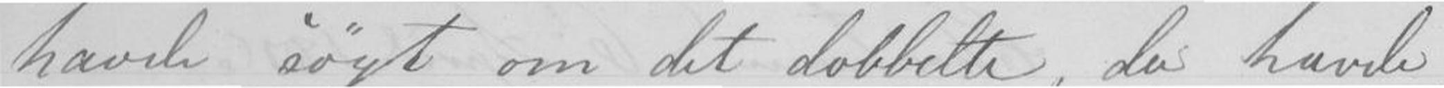havde søgt om det dobbelte, da havde
